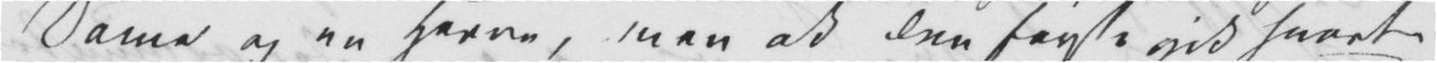Dame og en Herre, men ak den første gik snart
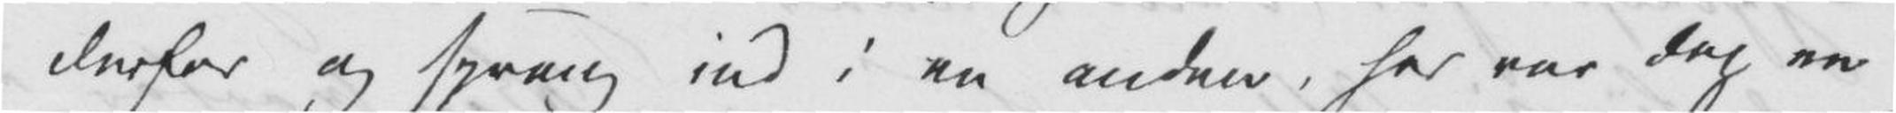derfor og sprang ind i en anden, her var dog en
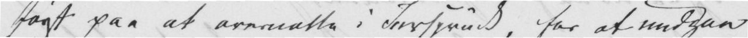først paa at overnatte i Innspruck, for at undgaa
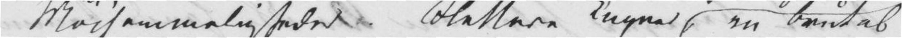Møisommeligheder. Slettere Kupeer, en brutal
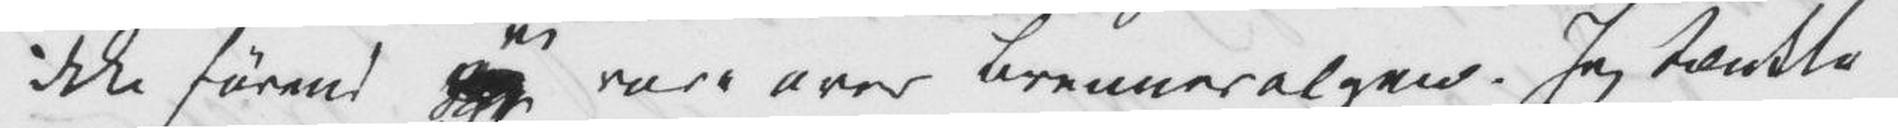ikke førend jeg var over Brenneralpene. Jeg tænkte
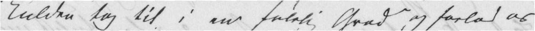Kulden tog til i en følelig Grad og forlod os
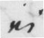vi
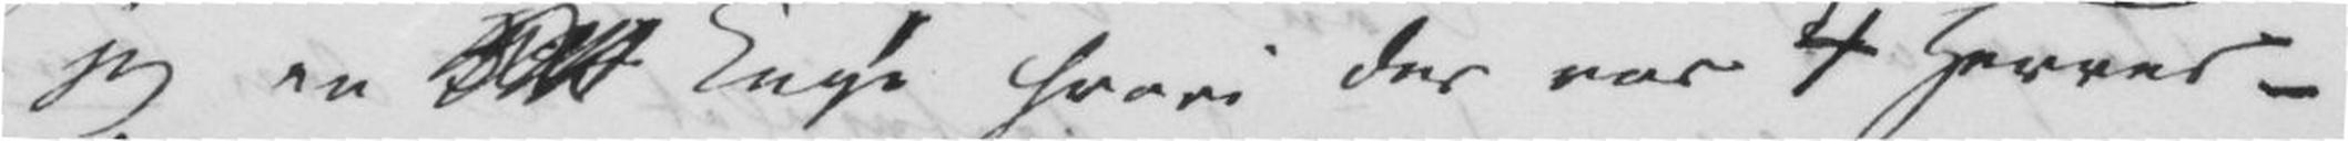jeg en <gap /></del> Kupé hvori der var 4 Herrer –
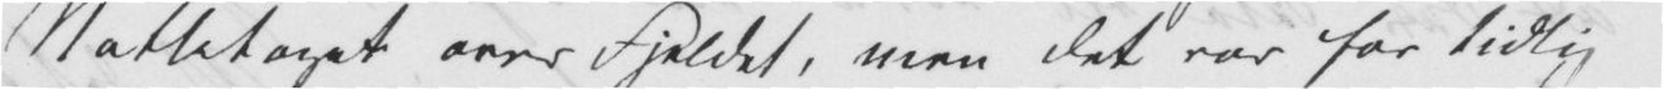Nattetoget over Fjeldet, men det var for tidlig
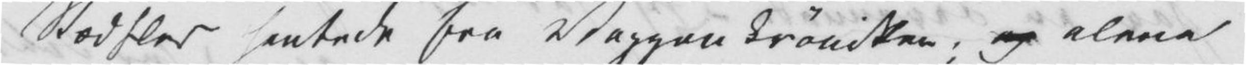Rædsler hentede fra Vaggon Krøniken; og alene
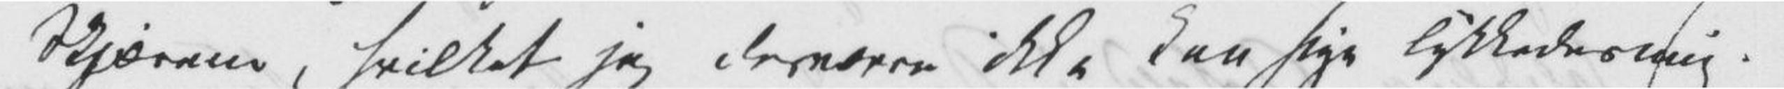Skjærene, hvilket jeg desværre ikke kan sige lykkedes mig.
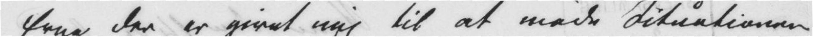Evne der er givet mig til at møde Situationen
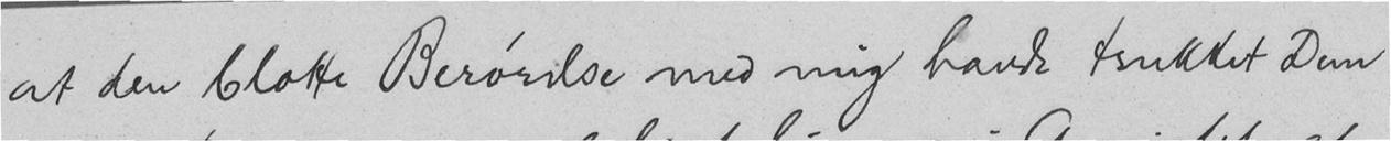at den blotte Berørelse med mig havde trukket Dem
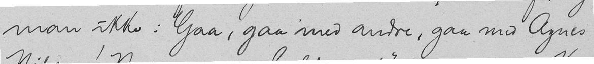man ikke: Gaa, gaa med andre, gaa med Agnes
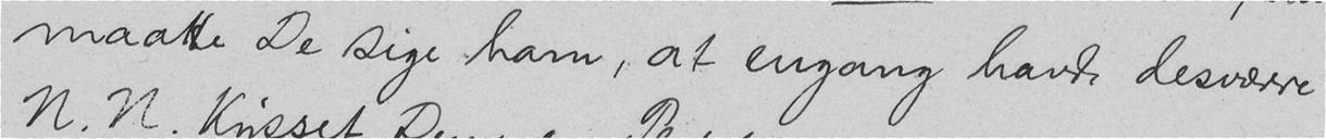maatte De sige ham, at engang havde desværre
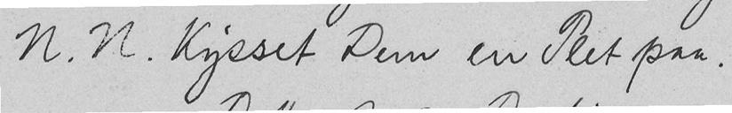N. N. kysset Dem en Plet paa.
